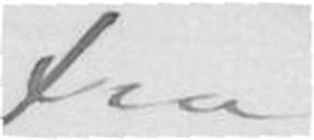fra
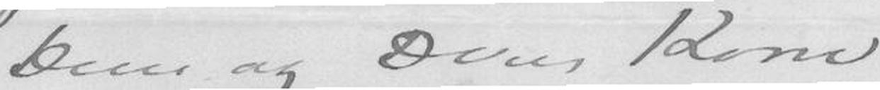Dem og Deres Kone
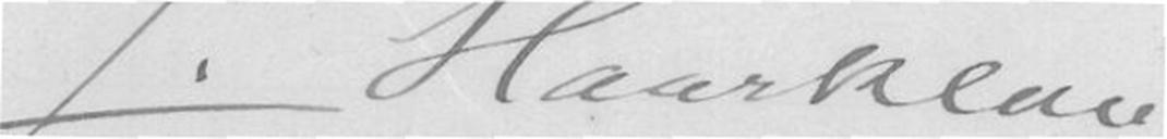J. Haarklou
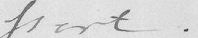svært.
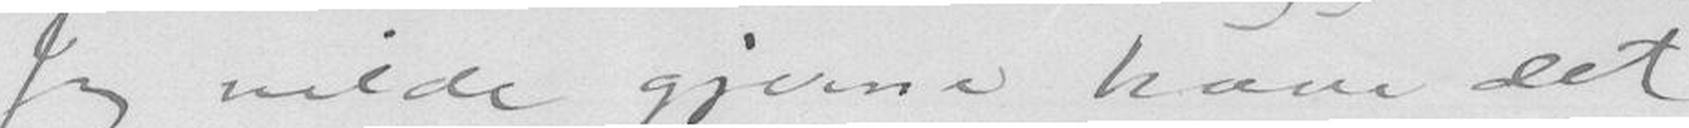Jeg vilde gjerne have det
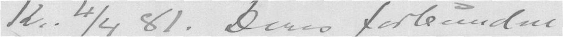Kr. 4/4 81. Deres forbundne
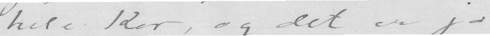hele Kor, og det er jo
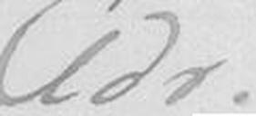Adr:
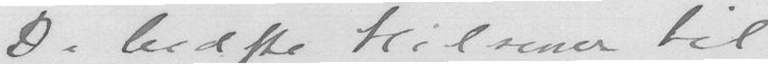De bedste Hilsener til
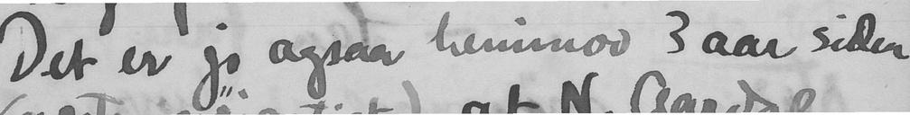Det er jo ogsaa henimod 3 aar siden
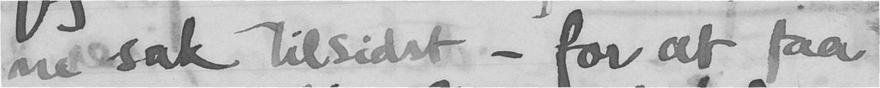ne sak tilsidst - for at faa
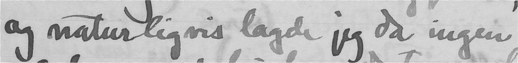og naturligvis lagde jeg da ingen
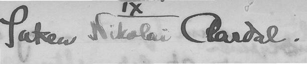Saken Nikolai Aardal.
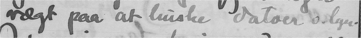vægt paa at huske datoer o. lgn.
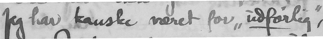Jeg har kanske været for "udførlig",
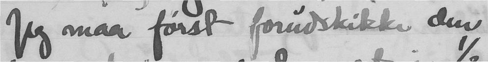Jeg maa først forudskikke den
